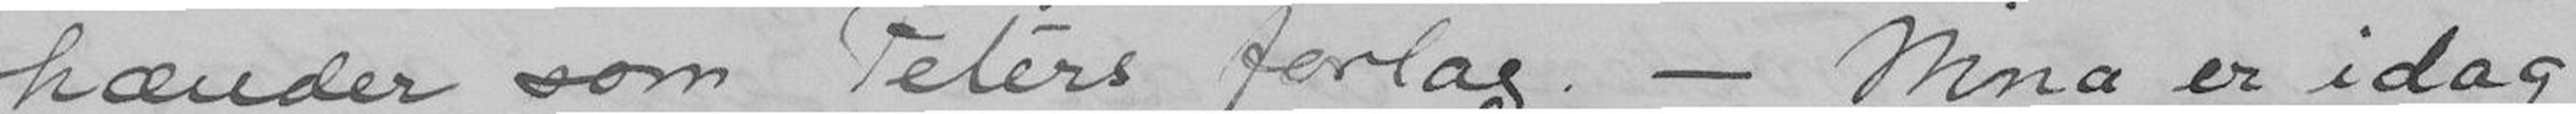hænder som Peters forlag. - Nina er idag
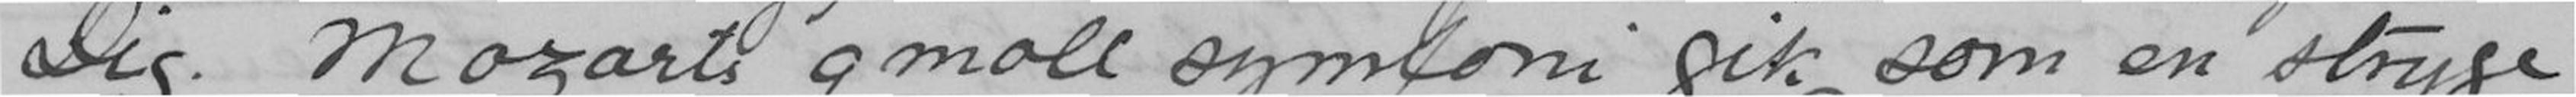Dig. Mozarts gmoll symfoni gik som en stryge
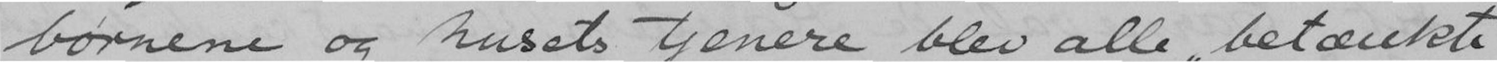børnene og husets tjenere blev alle betænkte
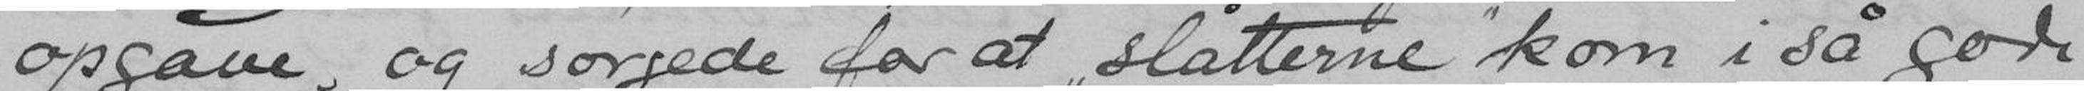opgave, og sørgede for at slåtterne kom i så gode
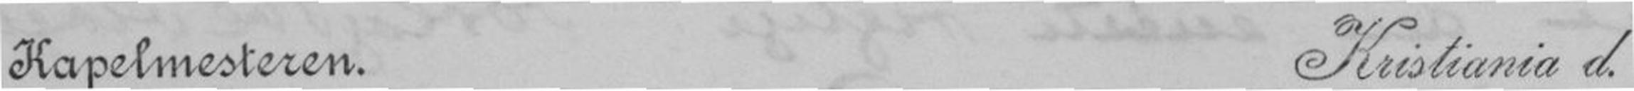Kapelmesteren. Kristiania d.
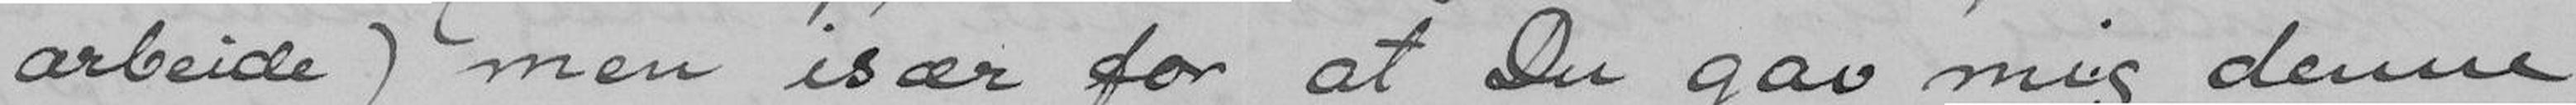arbeide) men især for at Du gav mig denne
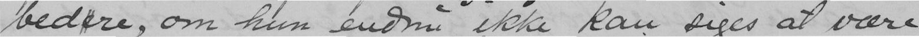bedre, om hun endnu ikke kan siges at være
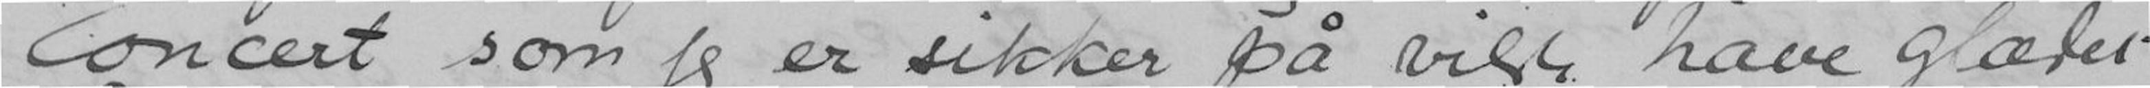concert som jeg er sikker på vilde have glædet
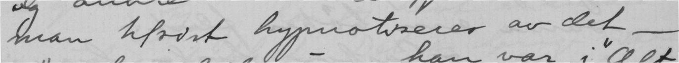man Ulødst hypnotiseres av det
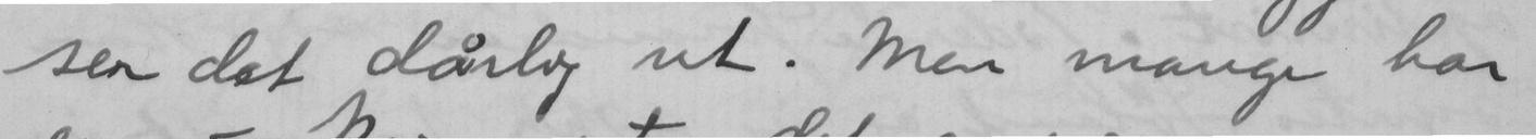ser det dårlig ut. Men mange har
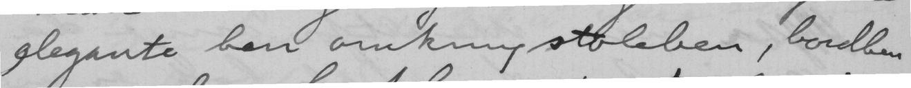elegante ben omkring stoleben, bordben
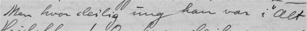Men hvor deilig ung han var i Alt
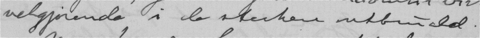velgjørende i de sterkere utbrudd.
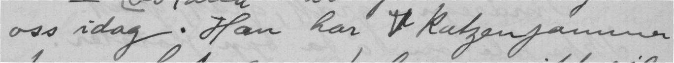oss idag. Han har  katzenjammer
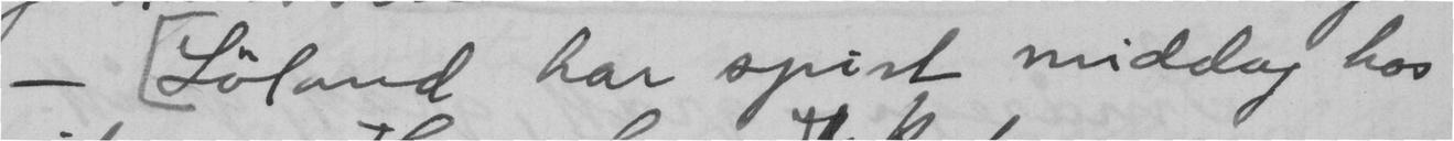- Løland har spist middag hos
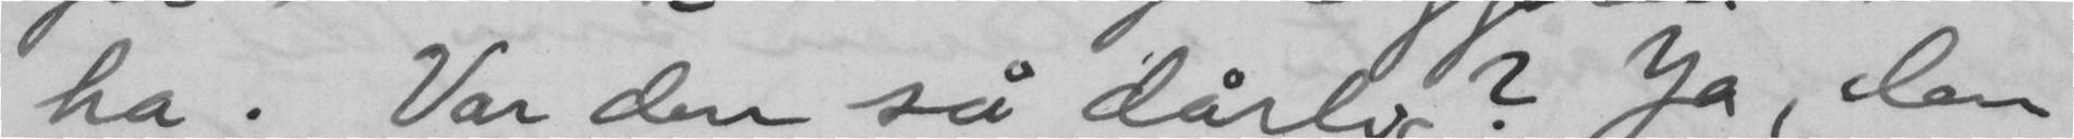ha. Var den så dårlig? Ja, den
